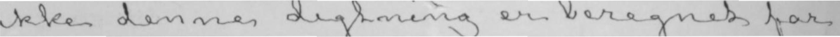ikke denne digtning er beregnet for
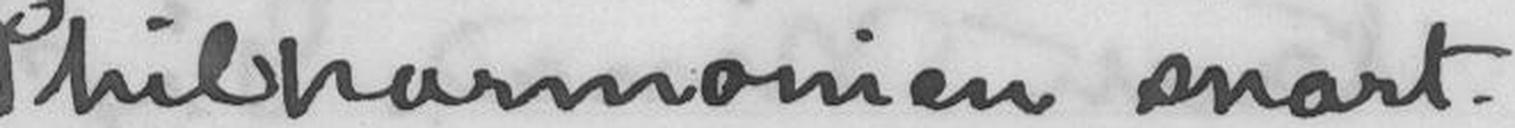Philharmonien snart.
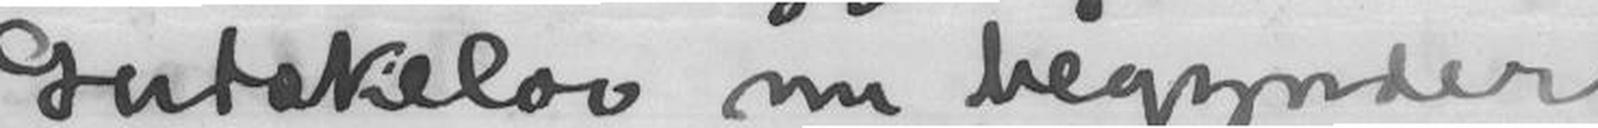Gutskelov nu begynder
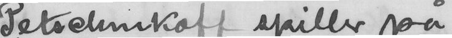Petschnikoff spiller på
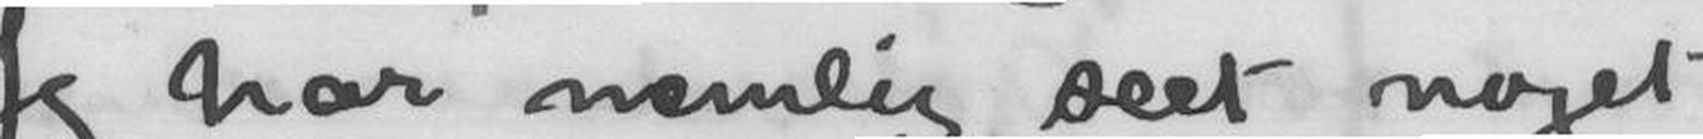Jeg har nemlig seet noget
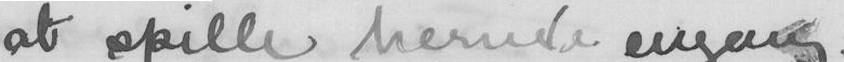at spille herude engang
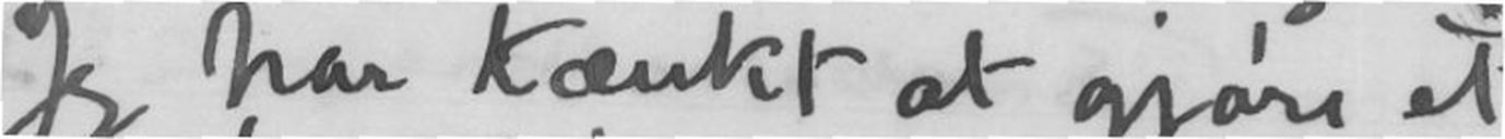Jeg har tænkt at gjøre et
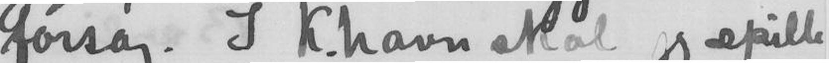forsøg. I K.havn skal jeg spille
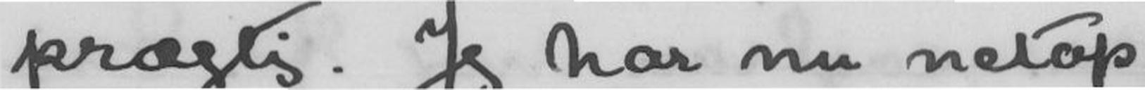prægtig. Jeg har nu netop
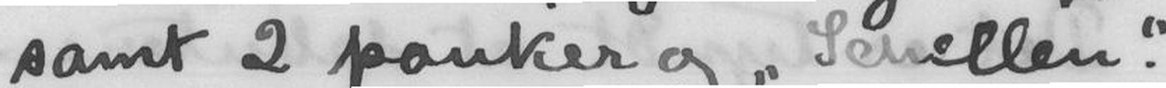samt 2 pauker og Schellen.
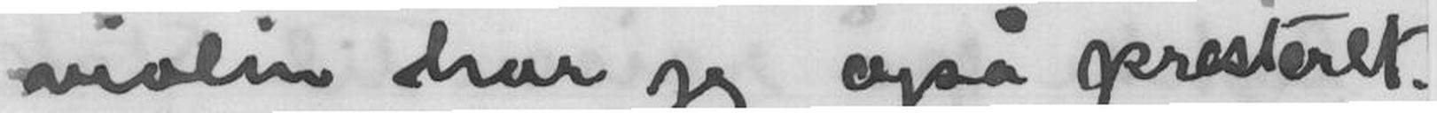violin har jeg også presteret.
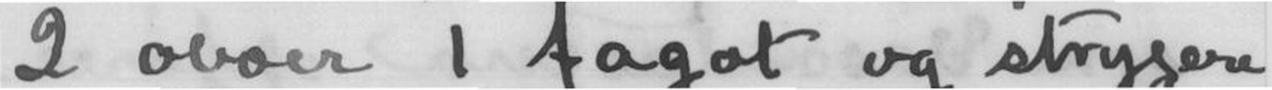2 oboer 1 fagot og strygere
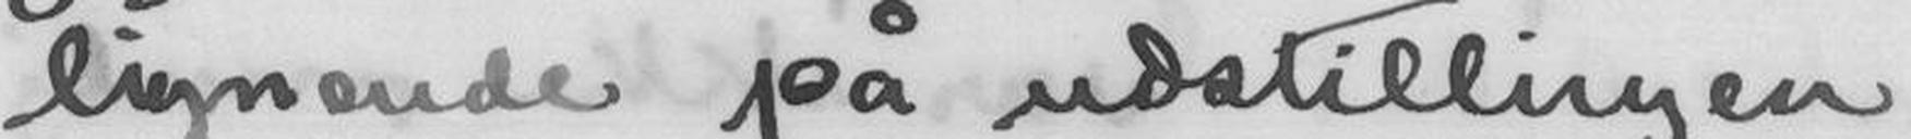lignende på udstillingen
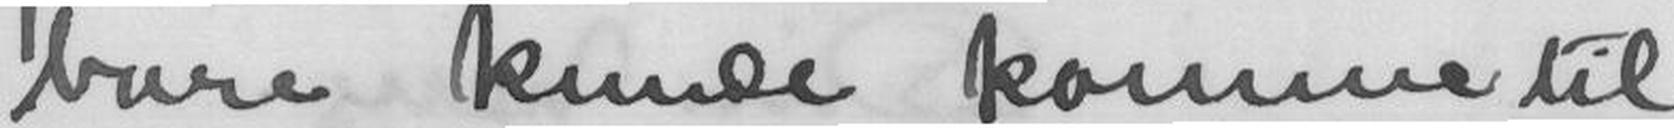bare kunde komme til
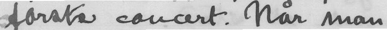første concert. Når man
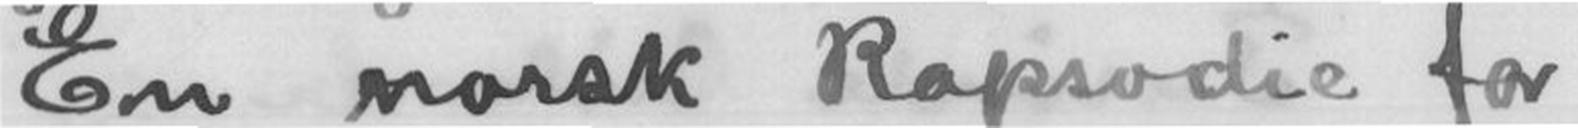En norsk Rapsodie for
